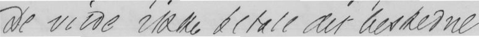De vilde ikke betale det beshedne
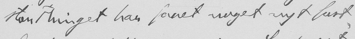storthinget har faaet noget nyt fast-
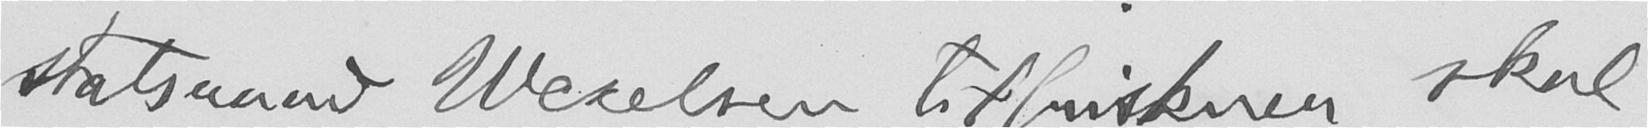statsraad Wexelsen tilfriskner, skal
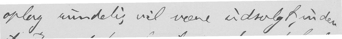oplag rundelig vil være udsolgt, inden
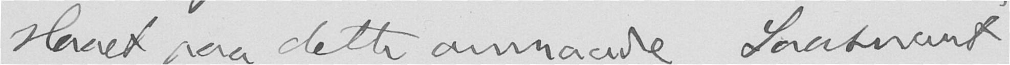slaaet paa dette omraade. Saasnart
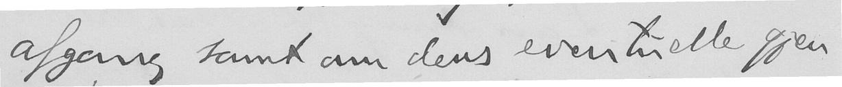afgang samt om dens eventuelle gjen-
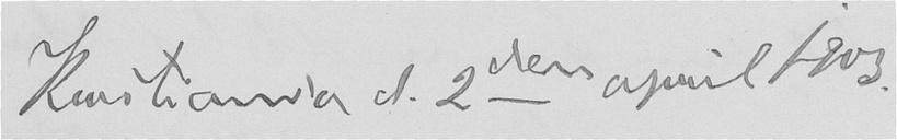Kristiania d. 2den april 1903.
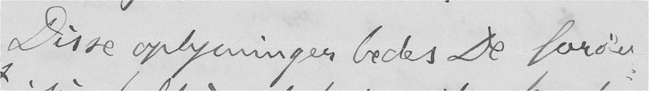Disse oplysninger bedes De forøv-
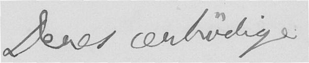Deres ærbødige
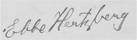Ebbe Hertzberg
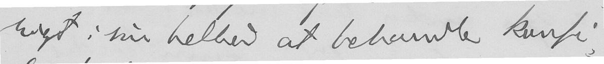rigt i sin helhed at behandle konfi-
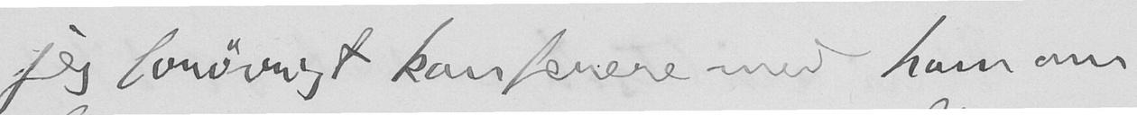jeg forøvrigt konferere med ham om
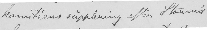komitéens supplering efter Storm's
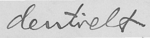dentielt.
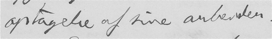optagelse af sine arbeider.
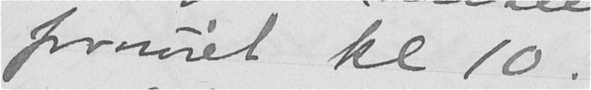fornøiet kl 10.
